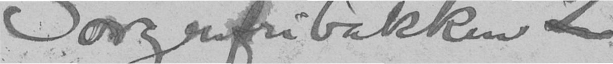Sorgenfribakken 2
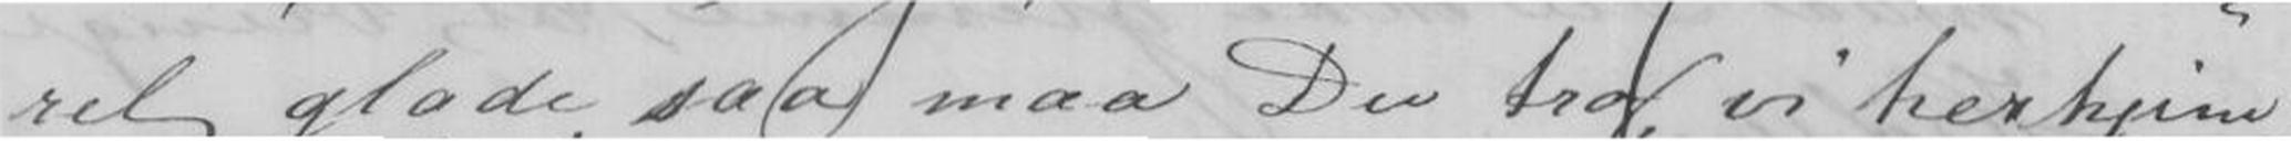ret glade, saa maa Du tro vi herhjem-
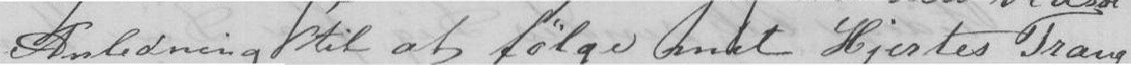Anledning til at følge mit Hjertes Trang
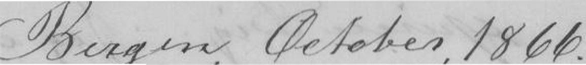Bergen, October, 1866.
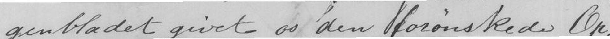genbladet givet os den forønskede Op-
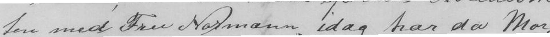ten med Fru Normann; idag har da Mor-
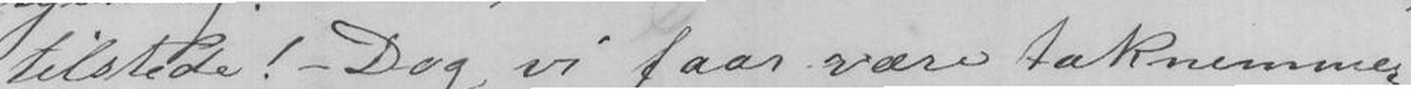tilstede! - Dog vi faar være taknemme-
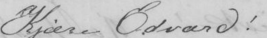Kjære Edvard!
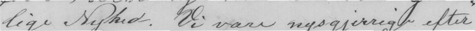lige Nyhed. Vi vare nygjerrige efter
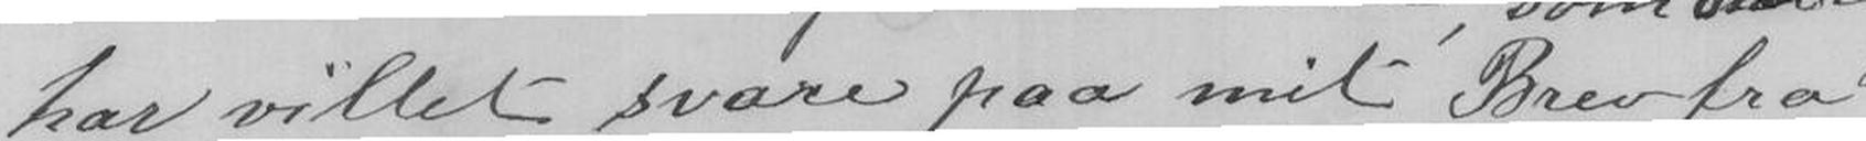har villet svare paa mit Brev fra
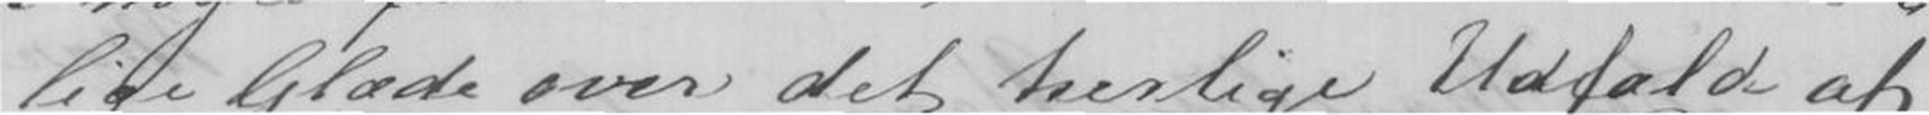lige Glæde over det herlige Udfald af
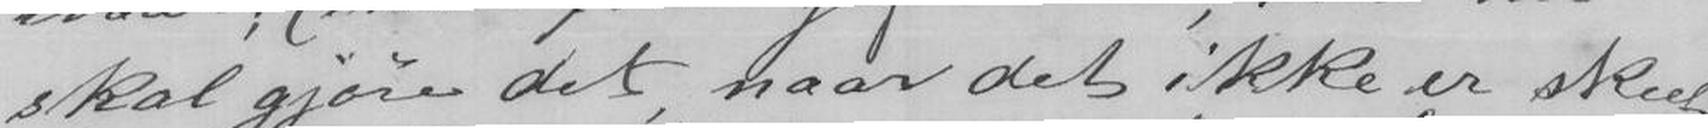skal gjøre det, naar det ikke er skeet
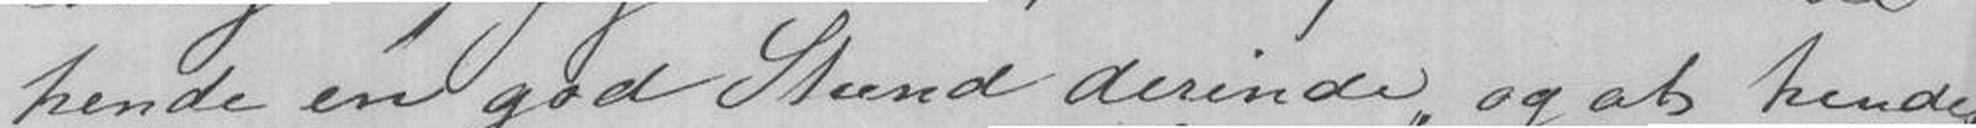hende en god Stund derinde, og at hendes
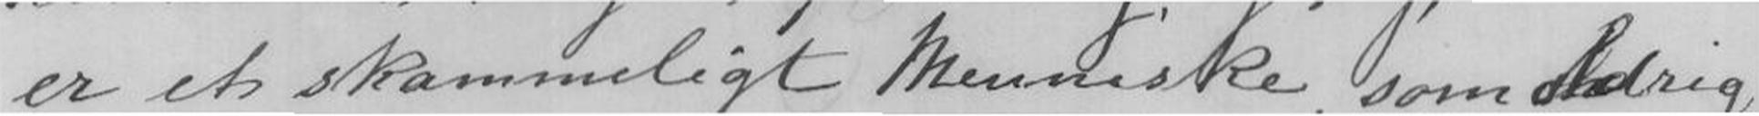er et skammeligt Menneske, som aldrig
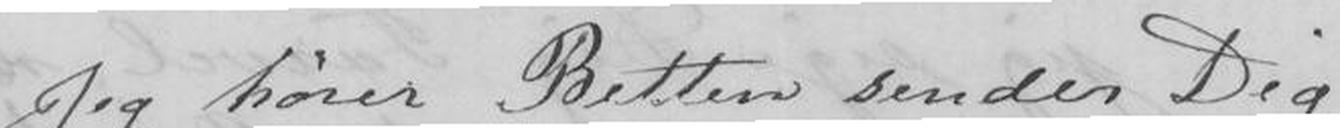Jeg hører Betten sender Dig
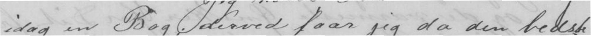idag en Bog; derved faar jeg da den bedste
